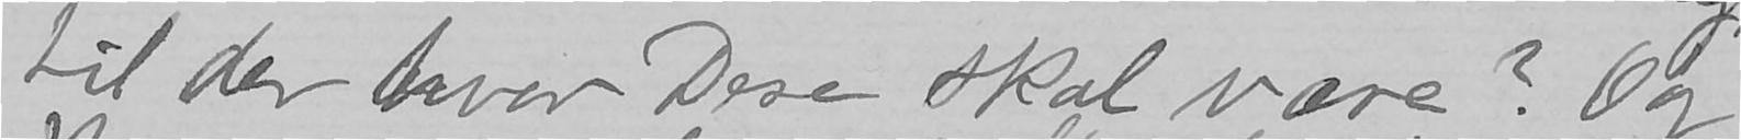til der hvor Dere skal være? Og
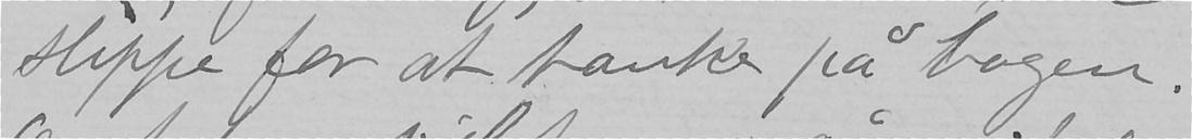slippe for at tænke på bogen.
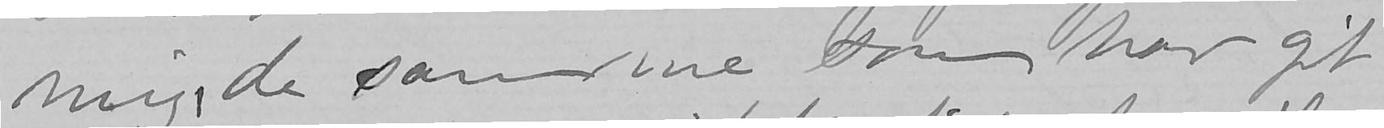mig, de samme som har git
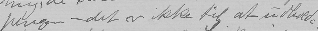penger - det er ikke til at udholde.
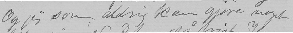Og jeg som aldrig kan gjøre noget
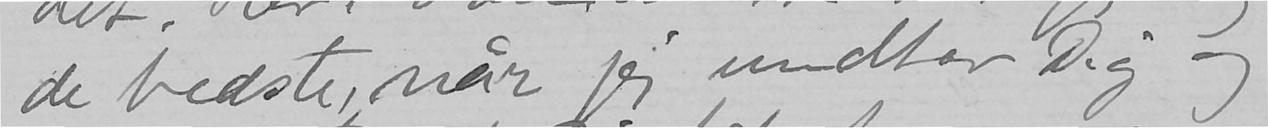de bedste, når jeg undtar Dig og
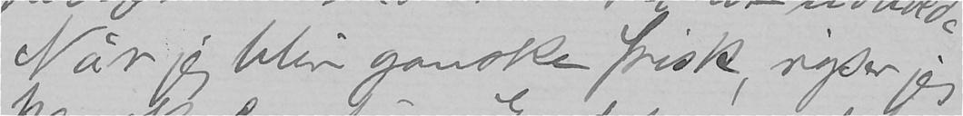Når jeg blir ganske frisk, rejser jeg
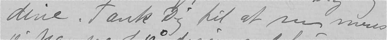dine. Tænk Dig til at nu mens
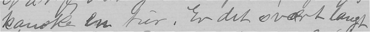kanske en tur. Er det svært langt
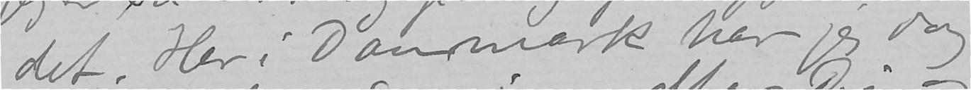det. Her i Danmark har jeg dog
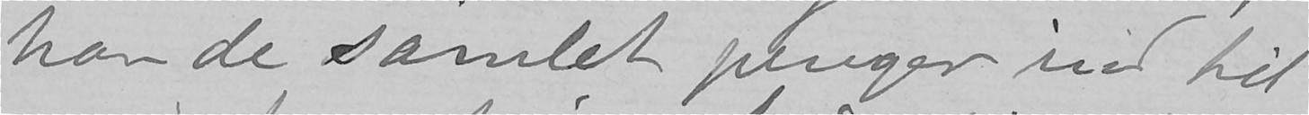har de samlet penger ind til
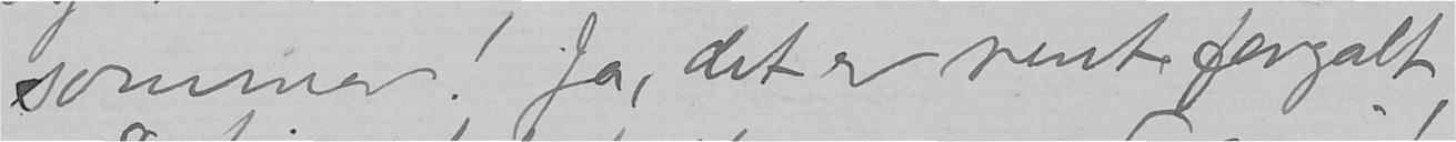sommer! Ja, det er rent forgalt
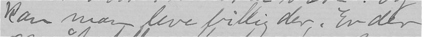kan man leve billig der. Er der
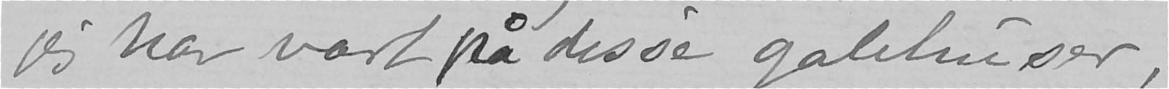jeg har vært på disse galehuser,
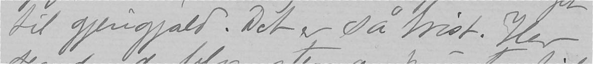til gjengjæld. Det er så trist. Her
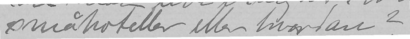småhoteller eller hvordan?
